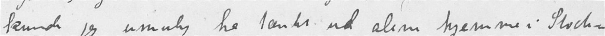kunde jeg umulig ha tænkt ud alene hjemme i Stock-
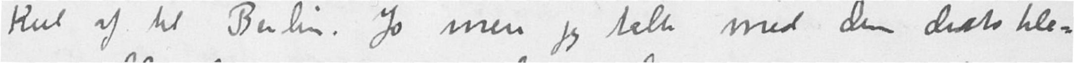Kiel og til Berlin. Jo mere jeg talte med dem desto kla-
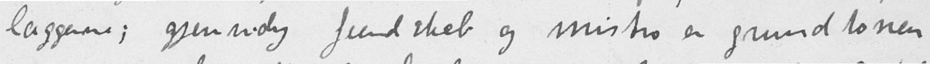læggerne; gjensidig fiendskab og mistro er grundtonen
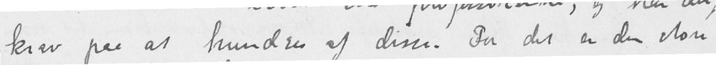krav paa at hundses af disse. For det er den store
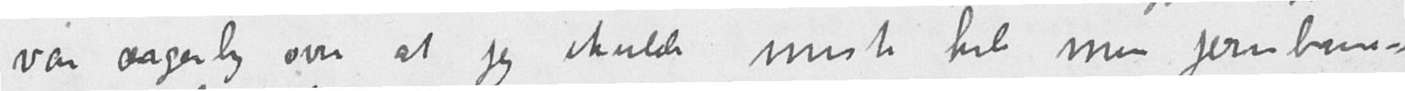var ærgerlig om at jeg skulde miste hele min jernbane-
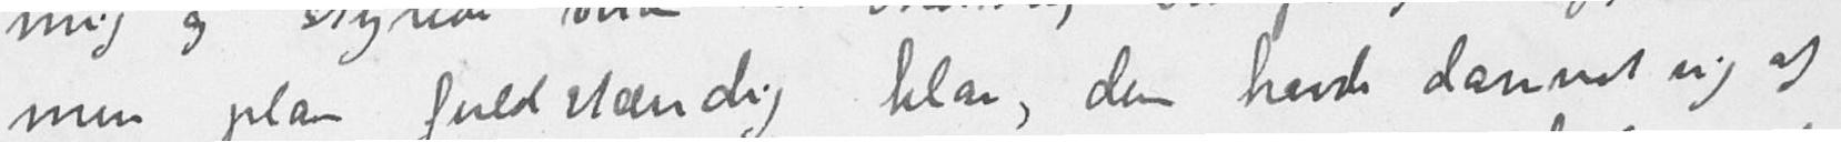min plan fuldstændig klar, den havde dannet sig af
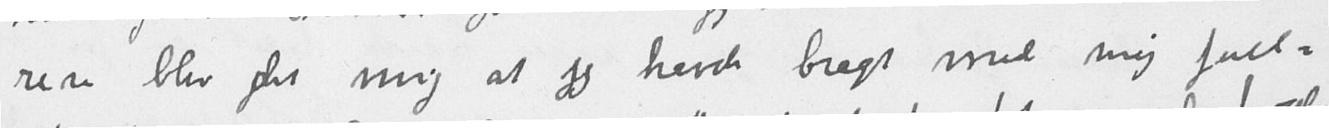rere blev det mig at jeg havde bragt med mig fuld-
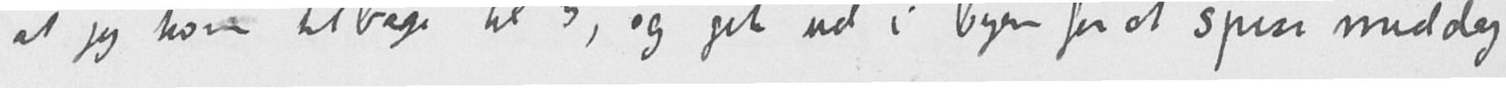at jeg kom tilbage kl 3, og gik ud i byen for at spise middag
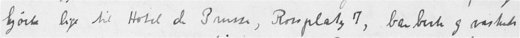kjørte lige til Hotel de Prusse, Rossplatz 7, barberte og vaskede
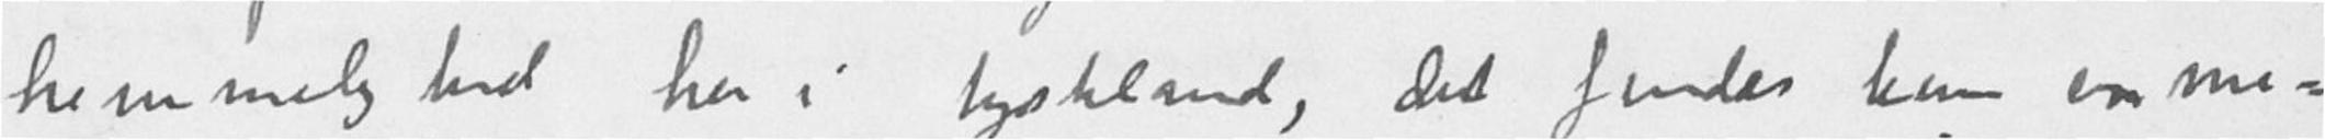hemmelighed her i tyskland, det findes kun en me-
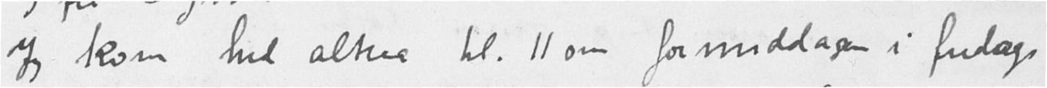Jeg kom hid altsaa kl. 11 om formiddagen i fredags
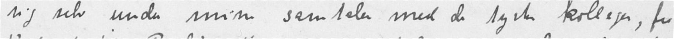sig selv under mine samtaler med de tyske kolleger, fra
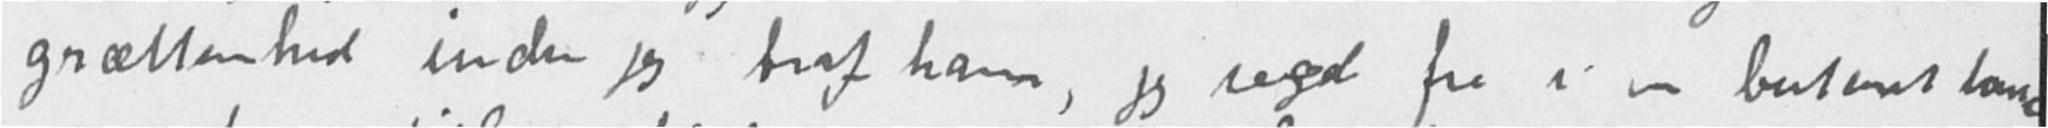grættenhed inden jeg traf ham, jeg sagde fra i en bestemt tone
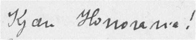Kjære Honoraria!
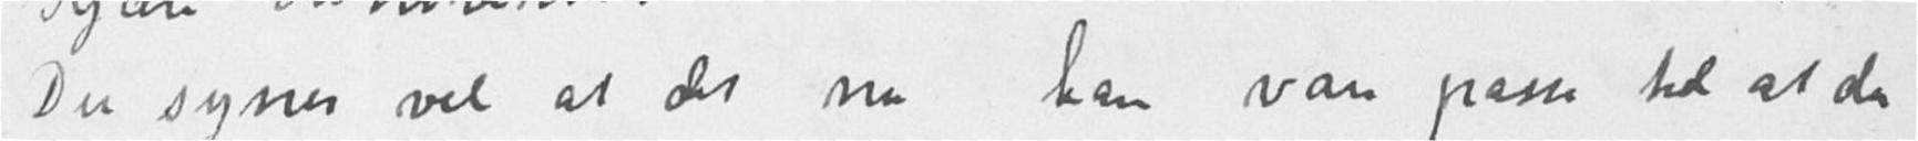Du synes vel at det nu kan være passe tid at du
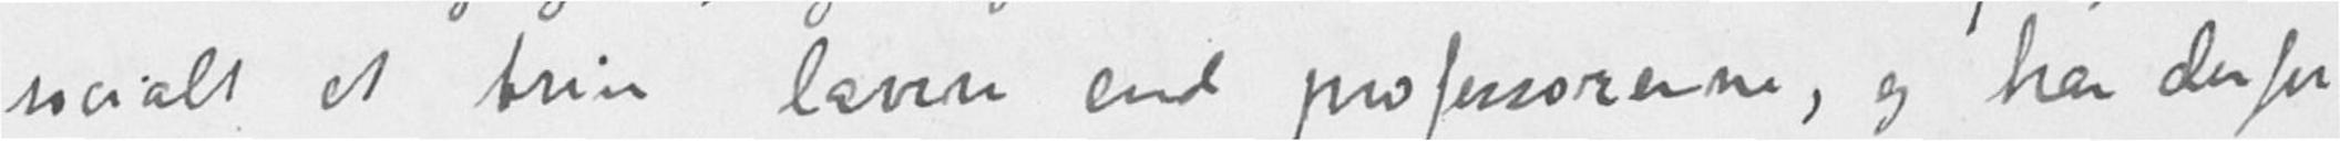socialt et trin lavere end professorerne, og har derfor
